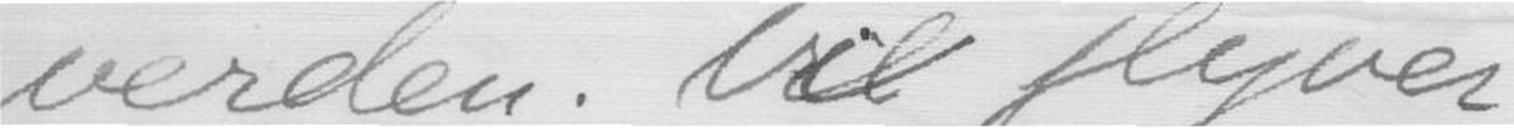verden. Vi flyver
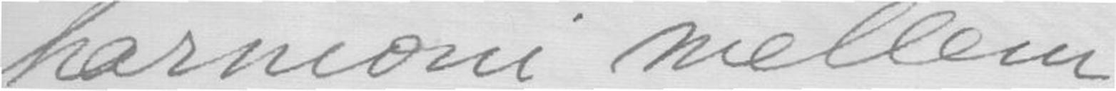harmoni mellem
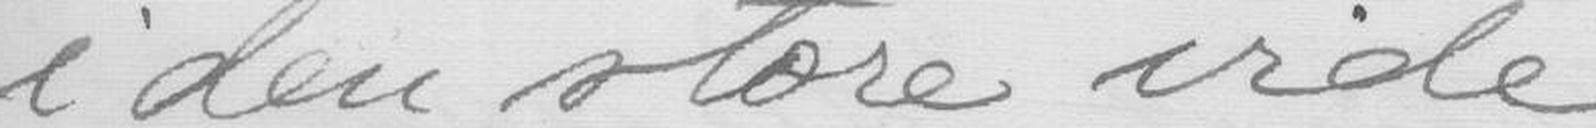i den store vide
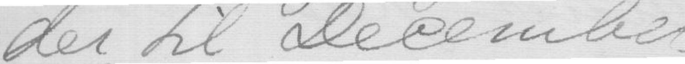der til December.
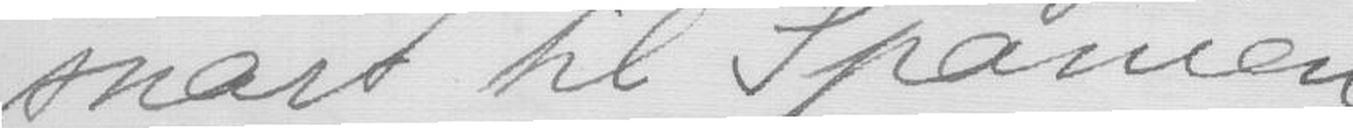snart til Spanien
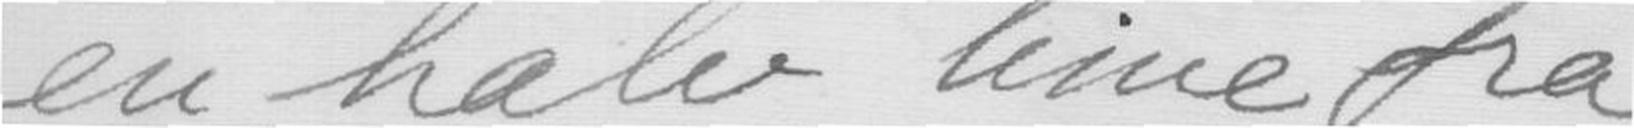en halv time fra
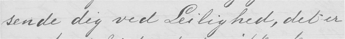sende dig ved Leilighed, det er
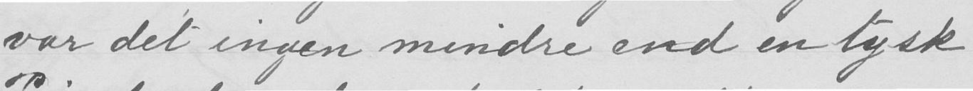var det ingen mindre end en tysk
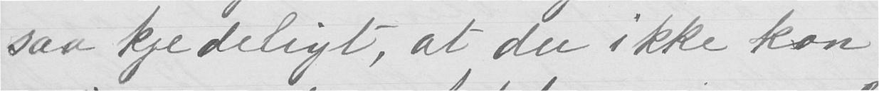saa kjedeligt, at du ikke kan
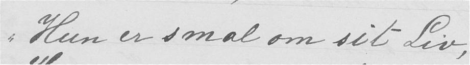"Hun er smal om sit Liv,
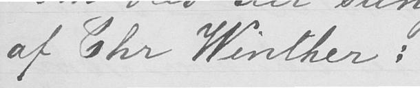af Chr Winther:
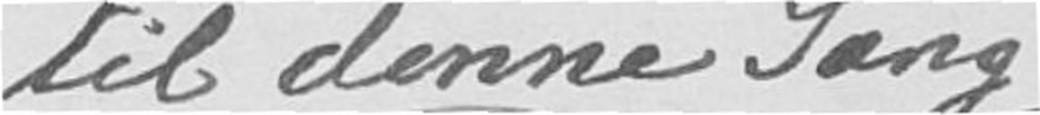til denne Sang,
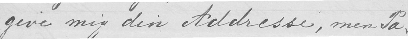give mig din Addresse, men Pa-
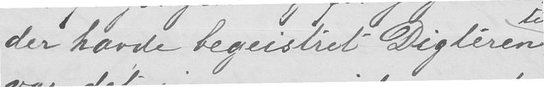der havde begeistret Digteren
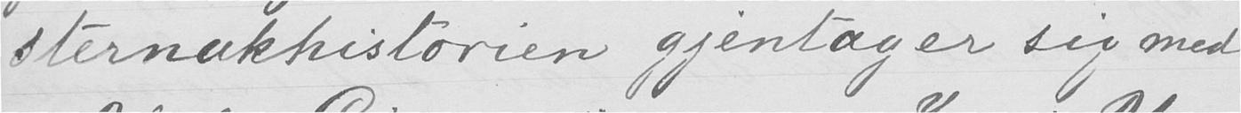sternakhistorien gjentager sig med
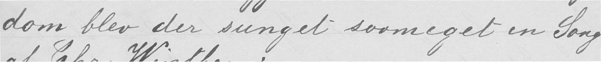dom blev der sunget saameget en Sang
